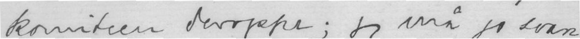komiteen deroppe; jeg må jo svare
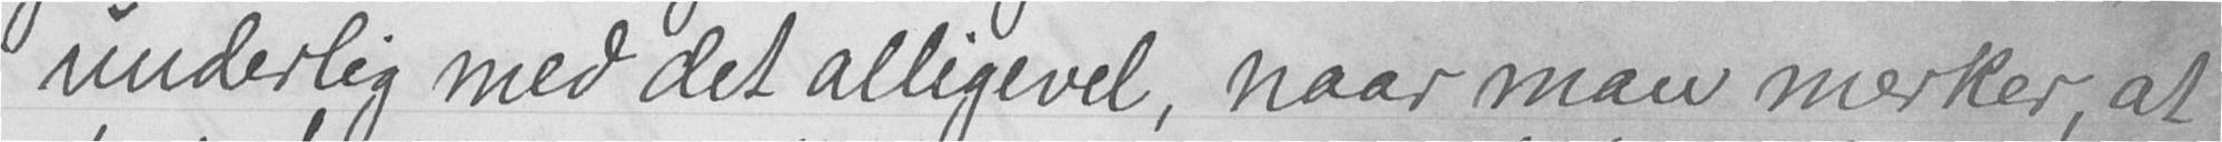underlig med det alligevel, naar man merker, at
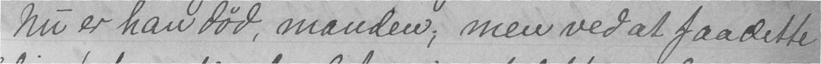Nu er han død, manden; men ved at faa dette
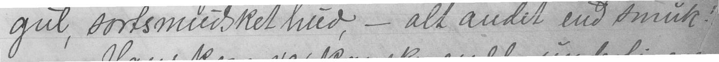gul, sortsmudsket hud, - alt andet end smuk!
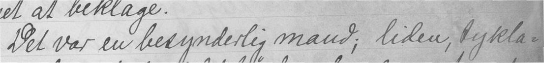Det var en besynderlig mand; liden, tykla-
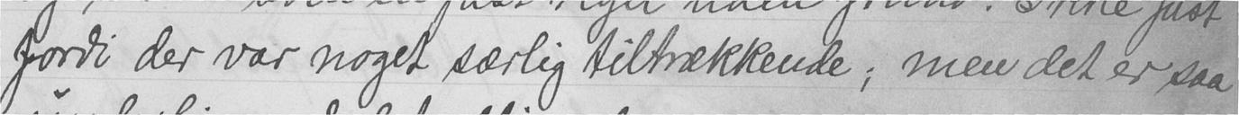fordi der var noget særlig tiltrækkende; men det er saa
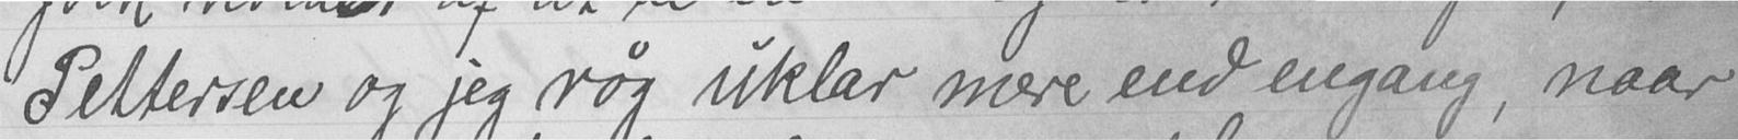Pettersen og jeg røg uklar mere end engang, naar
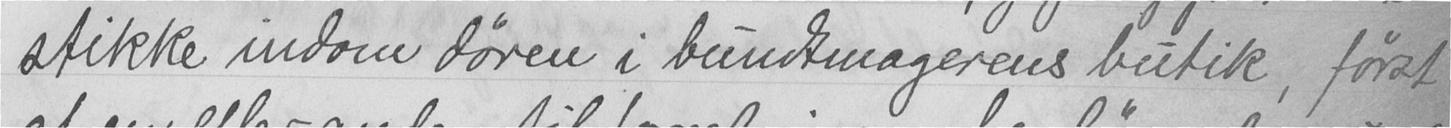stikke indom døren i bundtmagerens butik, først
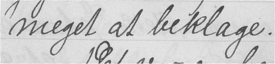meget at beklage.
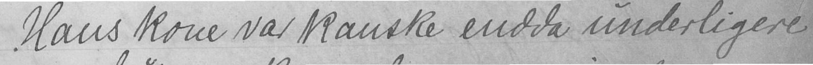Hans kone var kanske endda underligere
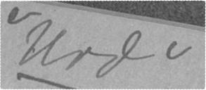"Urd"
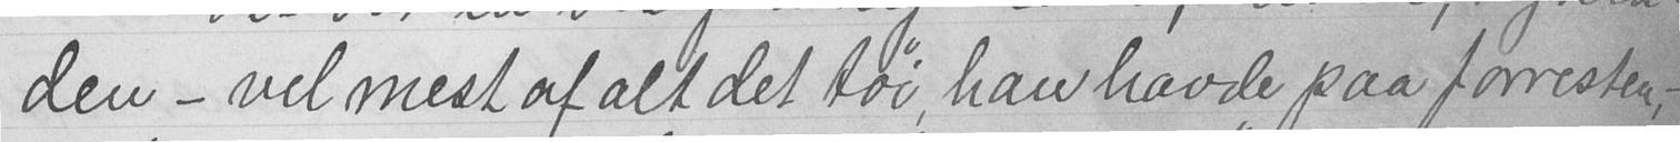den - vel mest af alt det tøi, han havde paa forresten, -
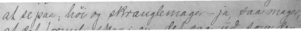at se paa; høi og skranglemager - ja, saa mager,
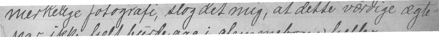merkelige fotografi, slog det mig, at dette værdige ægte-
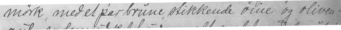mørk, med et par brune, stikkende øine og oliven-
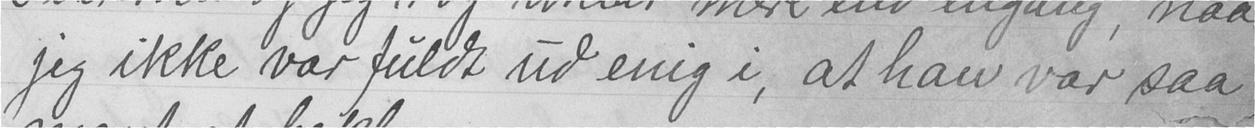jeg ikke var fuldt ud enig i, at han var saa
